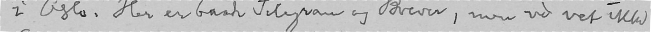i Oslo. Her er baade Telegram og Brever, men vi vet ikke
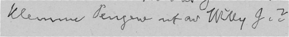klemme Pengene ut av Willy J.?
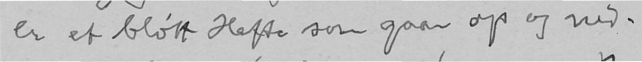er et bløtt Hefte som gaar op og ned.
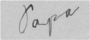Papa
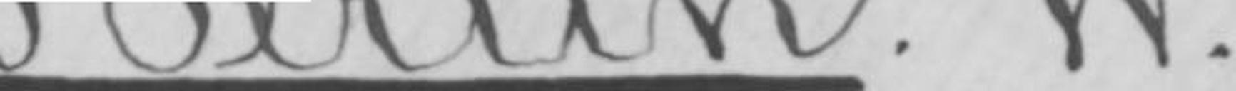Berlin. W.
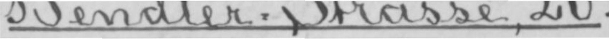Bendler-Strasse, 20.
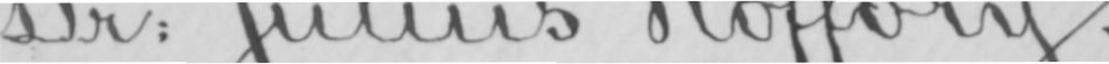Dr: Julius Hoffory.
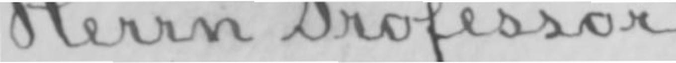Herrn Professor
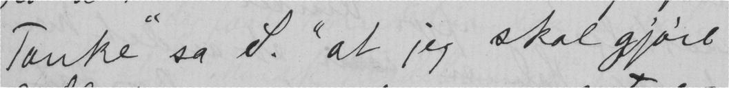Tanke" sa S. "at jeg skal gjøre
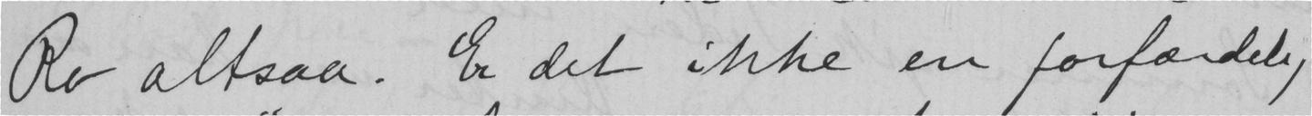Ro altsaa. Er det ikke en forfærdelig
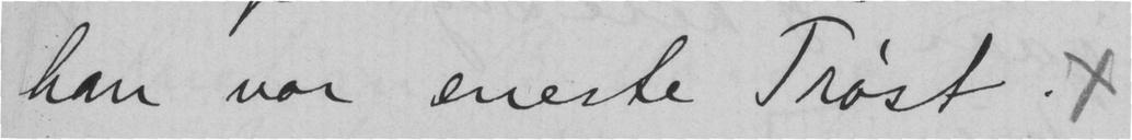han vor eneste Trøst. x
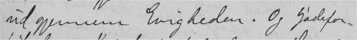ud gjennem Evigheden. Og jødefor-
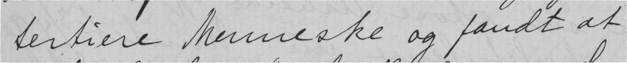tertiere menneske og fandt at
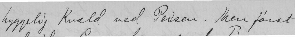hyggelig Kvæld ved Peisen. Men først
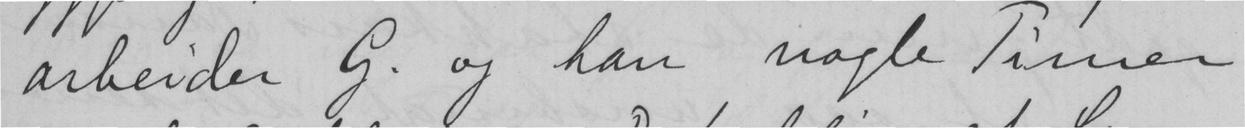arbeider G. og har nogle Timer
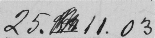25.11.03
